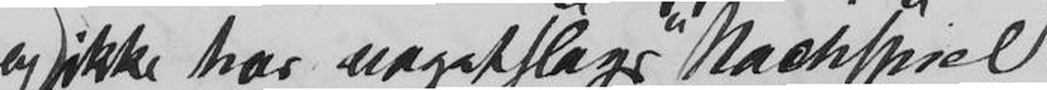og ikke har nogetslags Nachspiel
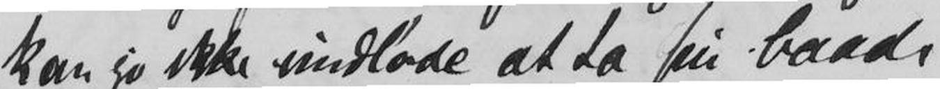kan jo ikke undlade at ta sin baade
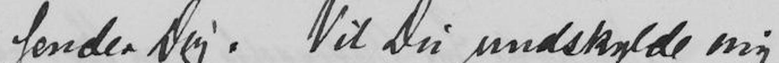sender Dig. Vil Du undskylde mig
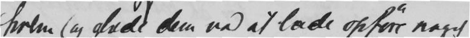holm og glæde dem ved at lade opføre noget
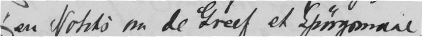en Notits om de Greef et Spørgsmaal,
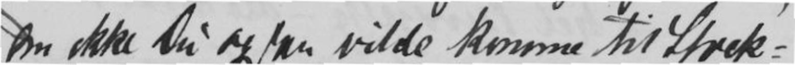om ikke Du ogsaa vilde komme til Stock-
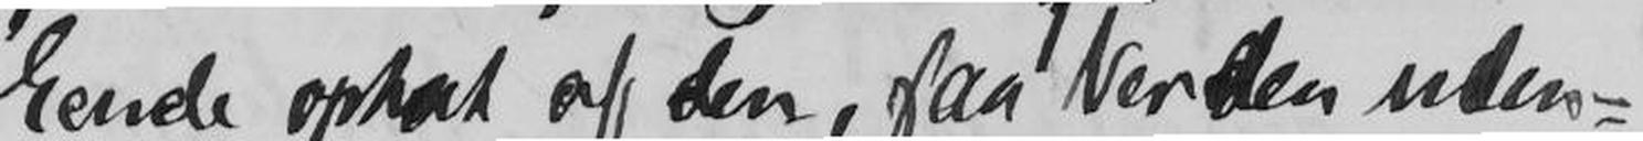kende optat af den, saa Verden uden-
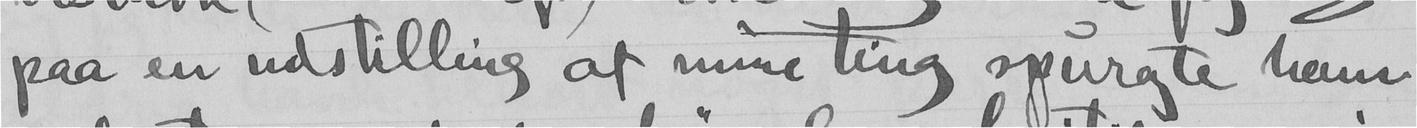paa en udstilling af mine ting spurgte ham
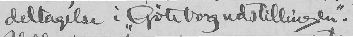deltagelse i "Gøteborg udstillingen".
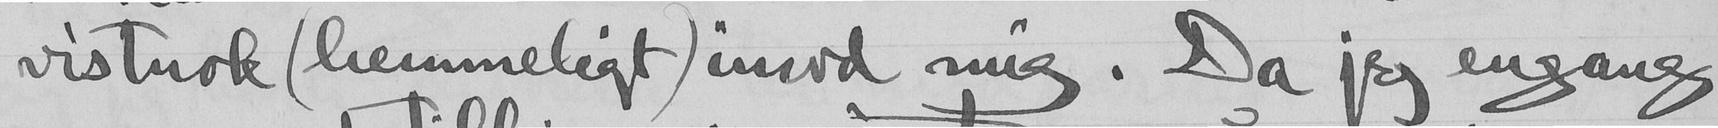vistnok (hemmeligt) imod mig. Da jeg engang
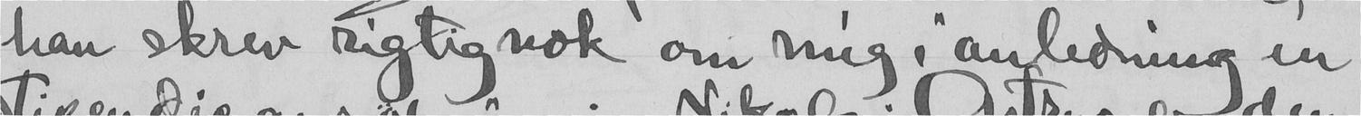han skrev rigtignok om mig i anledneng en
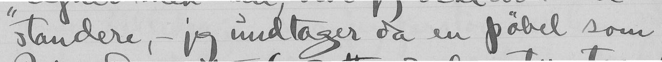standere, - jeg undtager da en pøbel som
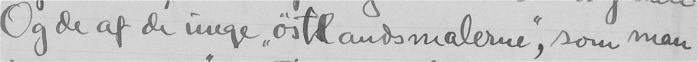Og de af de unge "østlandsmalerne", som man
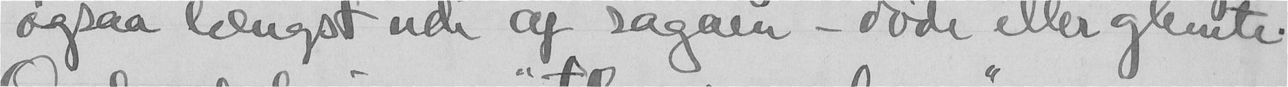ogsaa længst ude af sagaen - døde eller glemte.
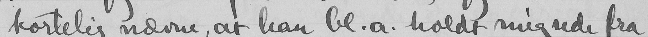kortelig nævne, at han bl.a. holdt mig ude fra
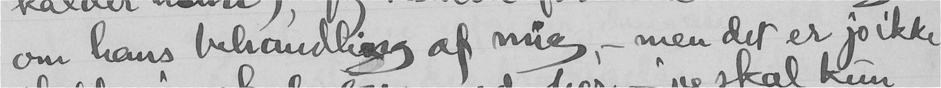om hans behandlig af mig, - men det er jo ikke
